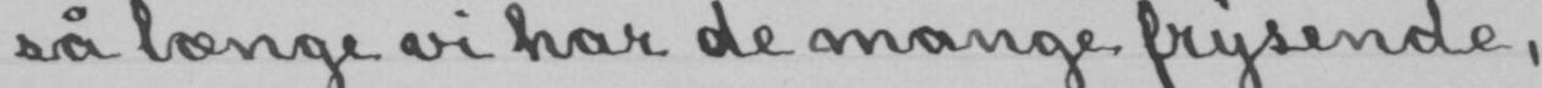så længe vi har de mange frysende,
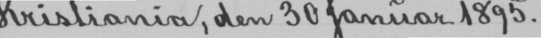Kristiania, den 30 Januar 1895.
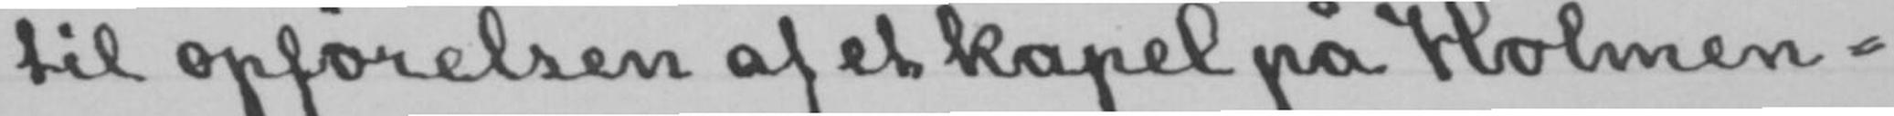til opførelsen af et kapel på Holmen-
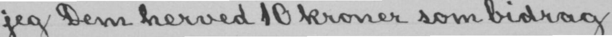jeg Dem herved 10 kroner som bidrag
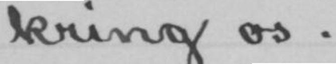kring os.
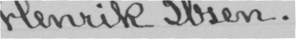Henrik Ibsen.
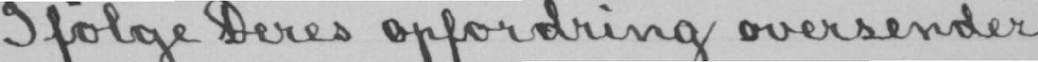Ifølge Deres opfordring oversender
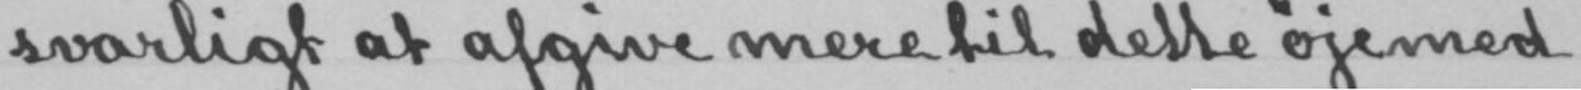svarligt at afgive mere til dette øjemed
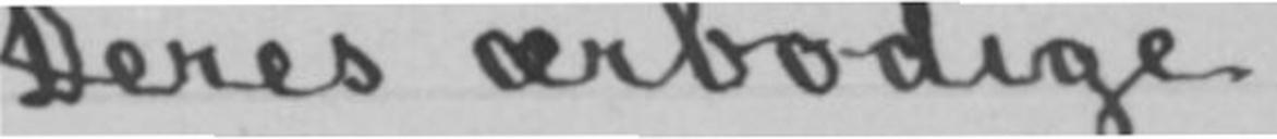Deres ærbødige
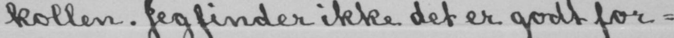kollen. Jeg finder ikke det er godt for-
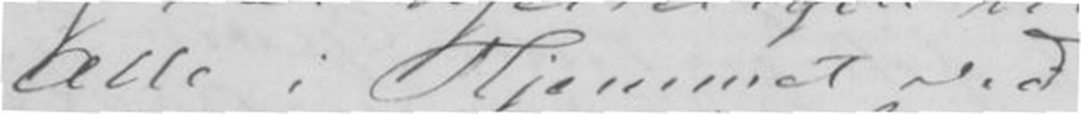Alle i Hjemmet ved
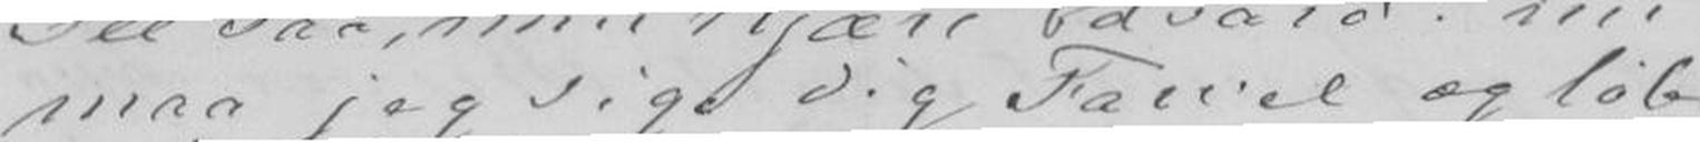maa jeg sige Dig Farvel og løbe
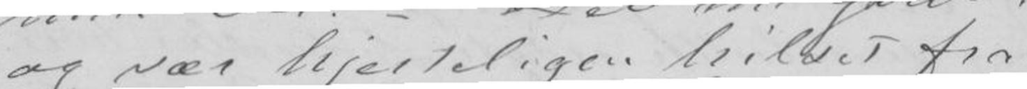og vær hjerteligen hilset fra
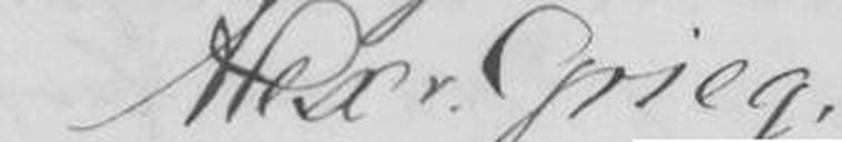Alex v. Grieg
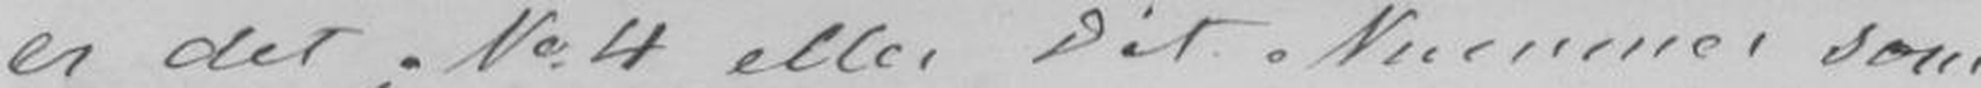er det No4 eller Dit Nummer som
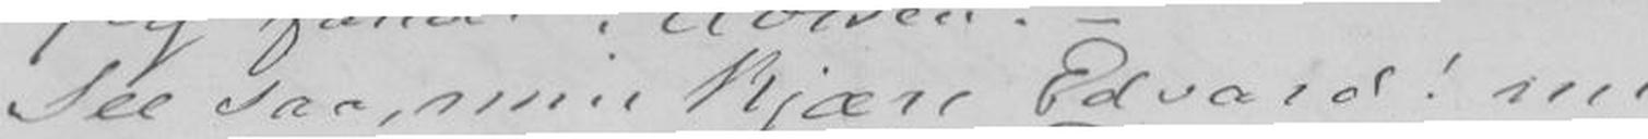See saa, min kjære Edvard! nu
